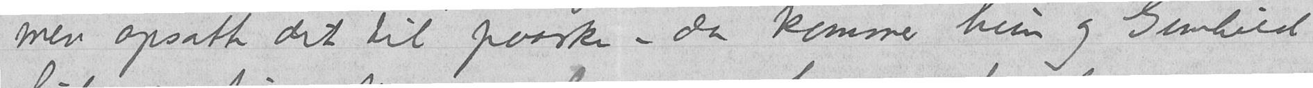men opsatte det til paaske – da kommer hun og Gunhild
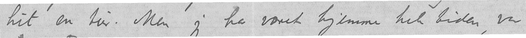hit en tur. Men jeg har været hjemme hele tiden, var
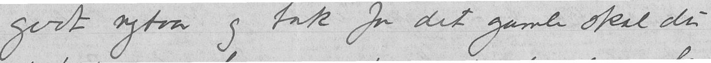godt nytaar og tak for det gamle skal du
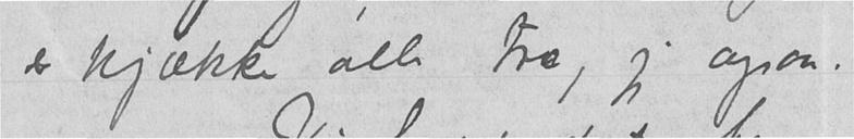er kjække alle tre, jeg ogsaa.
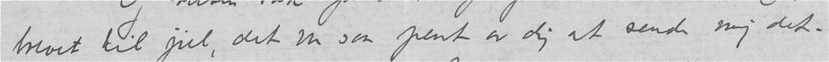brevet til jul, det var saa pent av dig at sende mig det.
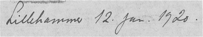Lillehammer 12. jan. 1920.
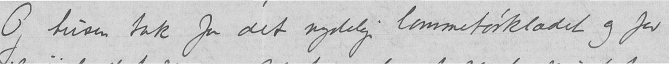Og tusen tak for det nydelige lommetørklædet og for
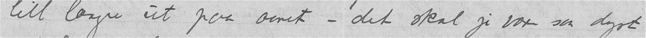litt længre ut paa aaret – det skal jo være saa dyrt
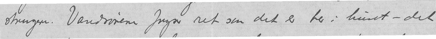strengere. Vandrørene fryser ret som det er her i huset – det
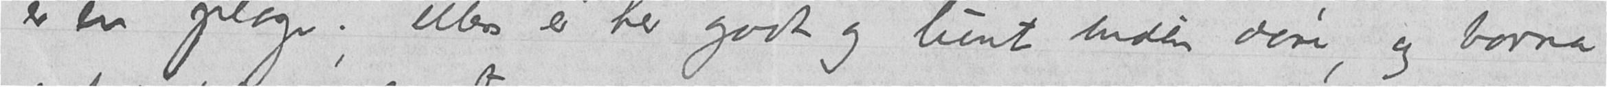er en plage; ellers er her godt og lunt inden døre, og barna
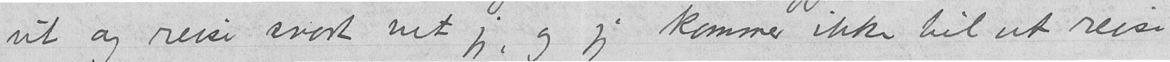ut og reise snart vet jeg, og jeg kommer ikke til at reise
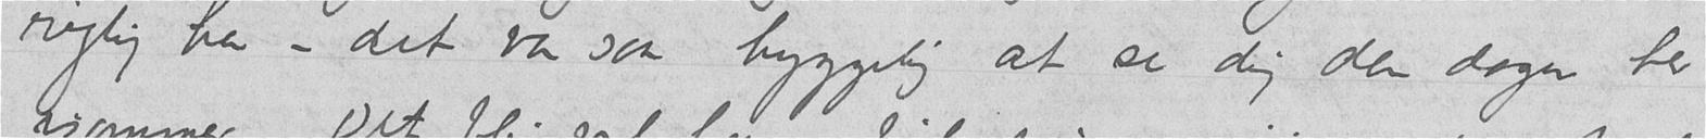rigtig ha – det var saa hyggelig at se dig den dagen her
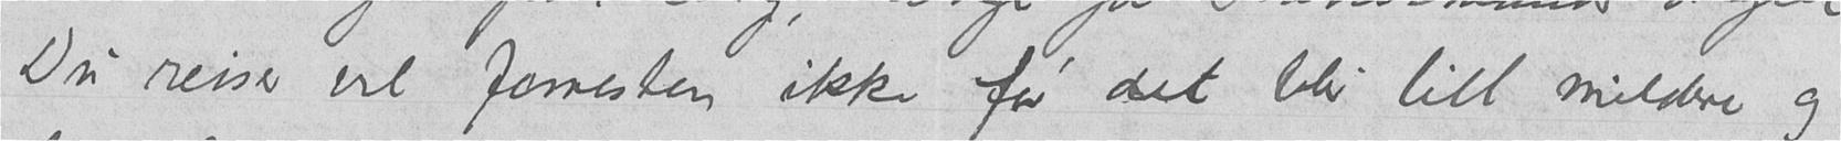Du reiser vel forresten ikke før det blir litt mildere og
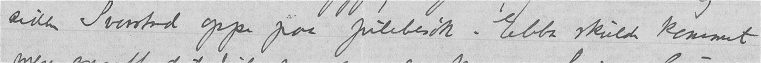siden Svarstad oppe paa julebesøk – Ebba skulde kommet
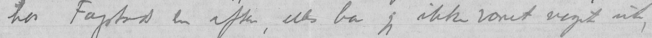hos Fagstads en aften, ellers har jeg ikke været noget ute,
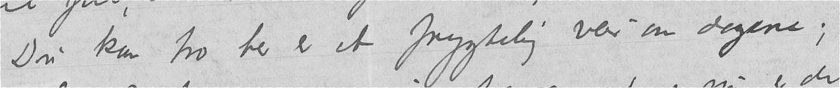Du kan tro her er et frygtelig veir om dagene;
